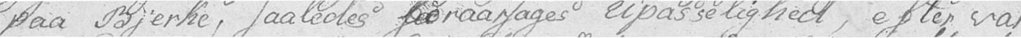paa Bjerke, saaledes foraarsages upasselighed, efter var
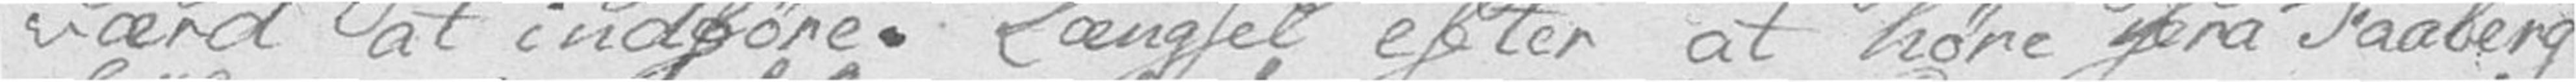værd at indføre. Længsel efter at høre fra Faaberg
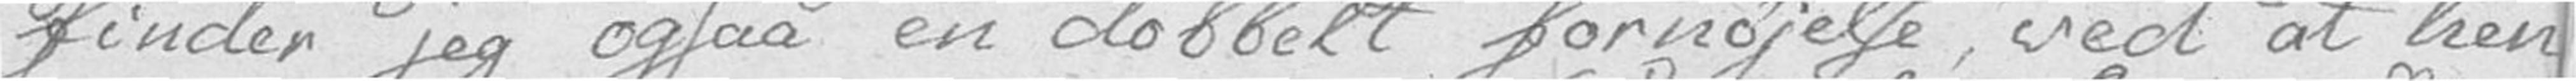finder jeg ogsaa en dobbelt fornøjelse, ved at hen
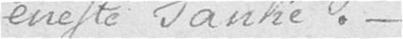eneste Tanke. –
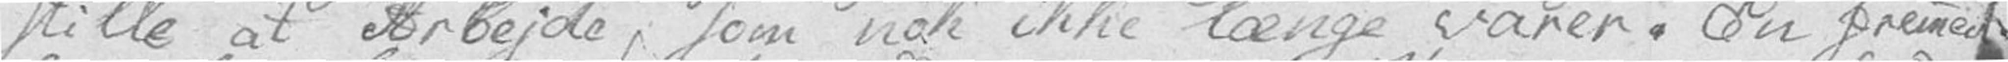stille at Arbejde, som nok ikke længe varer. En fremmed
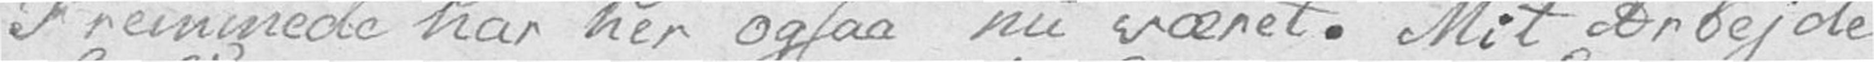Fremmede har her ogsaa nu været. Mit Arbejde
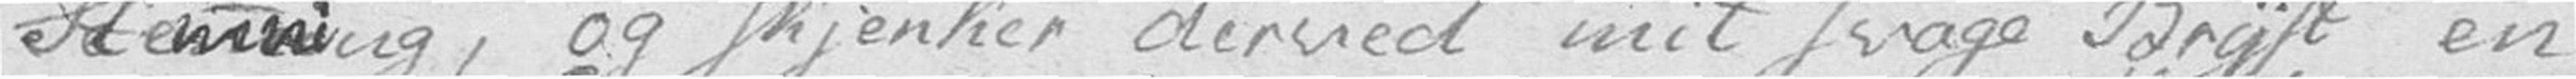Stemning, og skjenker derved mit svage Bryst en
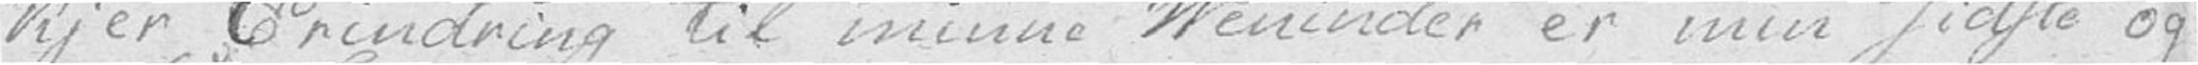kjer Erindring til minne Veninder er min sidste og
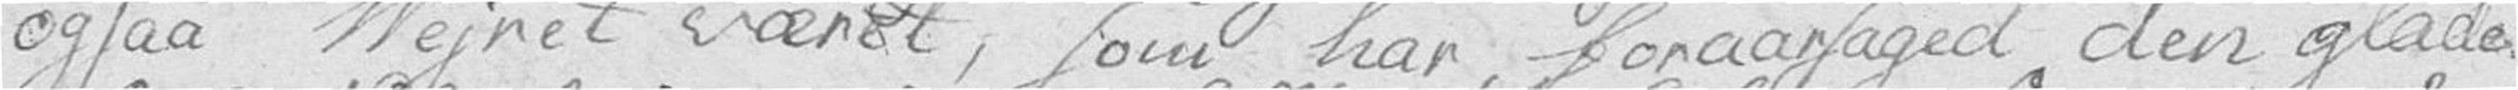ogsaa Vejret været, som har foraarsaged den glade
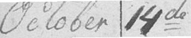October 14de
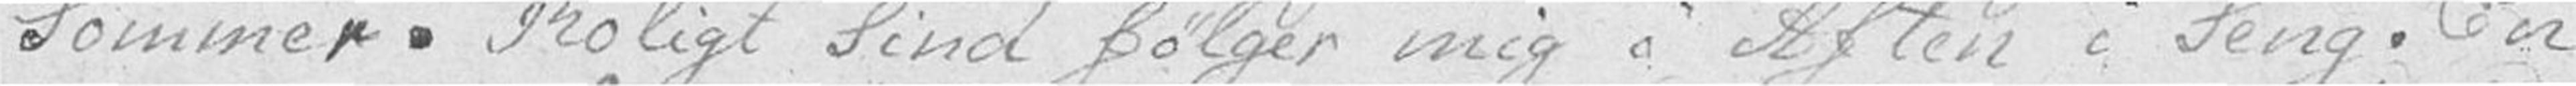Sommer. Roligt Sind følger mig i Aften i Seng. En
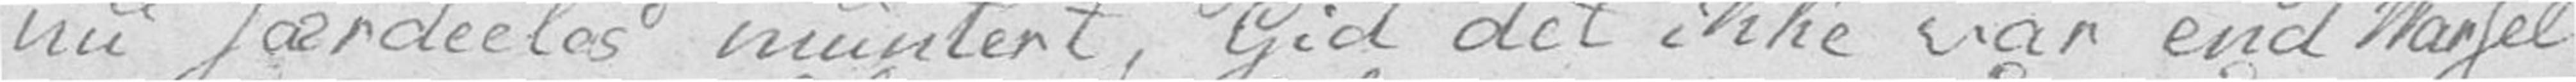nu særdeeles muntert, Gid det ikke var end Varsel
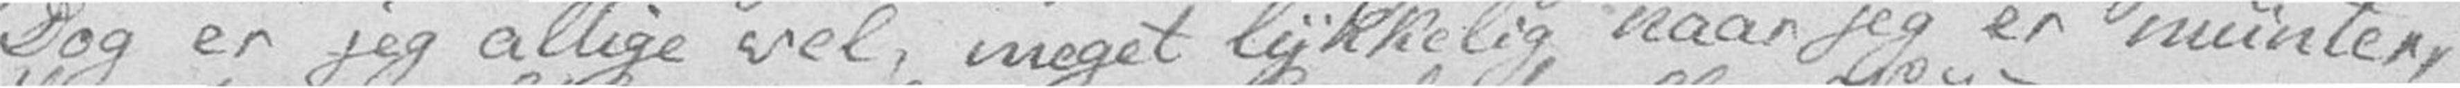Dog er jeg allige vel, meget lykkelig naar jeg er munter,
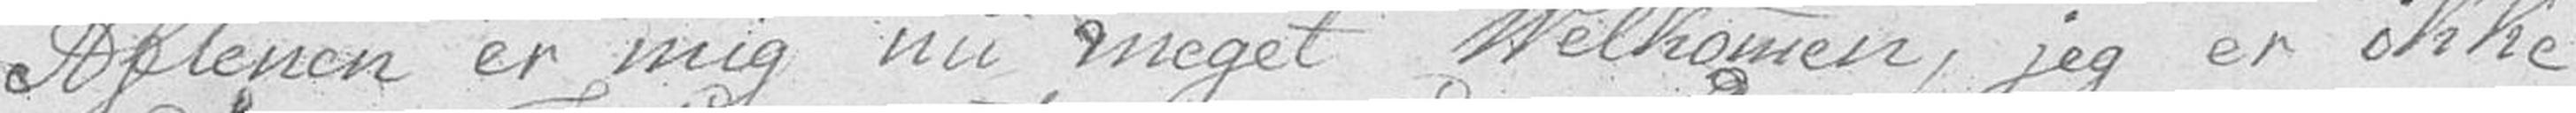Aftenen er mig nu meget Velkomen, jeg er ikke
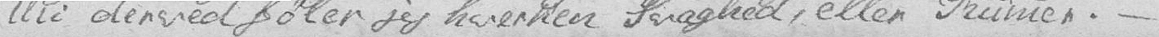thi derved føler jeg hverken Svaghed, eller Kummer. –
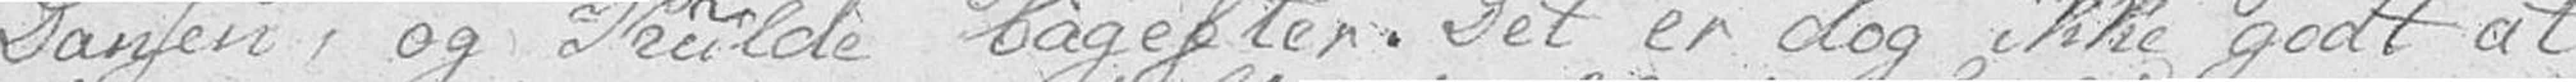Dansen, og Kulde bagefter. Det er dog ikke godt at
